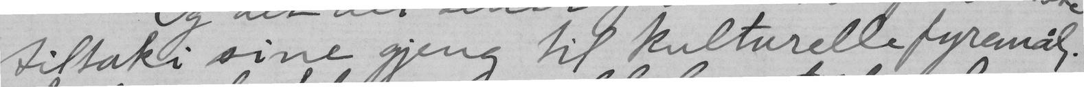tiltaki sine gjeng til kulturelle fyremål.
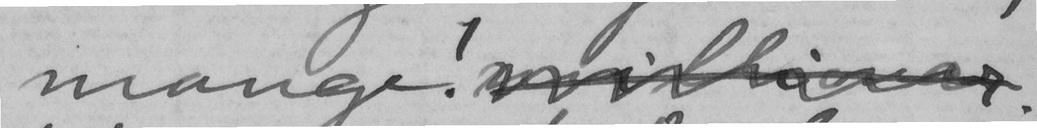mange! millionar.
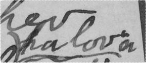ha lov å
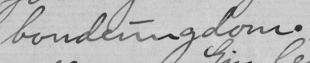bondeungdom.
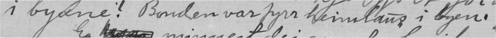i byane! Bonden var fyrr heimlaus i byen.
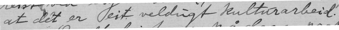at det er eit veldigt kulturarbeid.
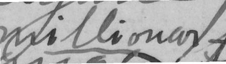millionar
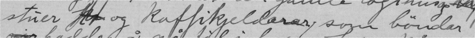stuer fr og kaffikjeldarar, som bønder
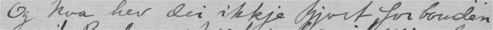Og kva hev dei ikkje gjort for bonden
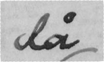då
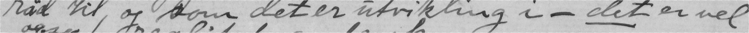råd til, og som det er utvikling i - det er vel
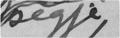segje,
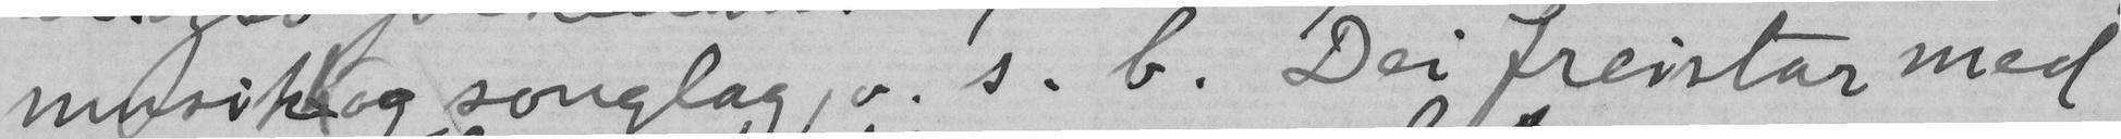musik og songlag, o. s. b. Dei freistar med
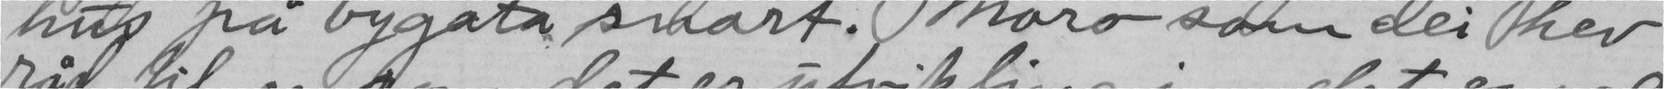hus på bygata snart. Moro som dei hev
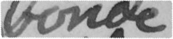bonde
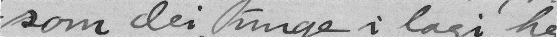som dei unge i lagi hev
